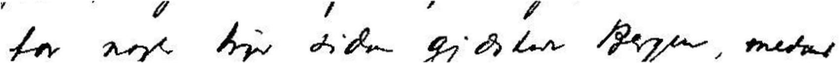for nogle Uger siden gjæstede Bergen, medend
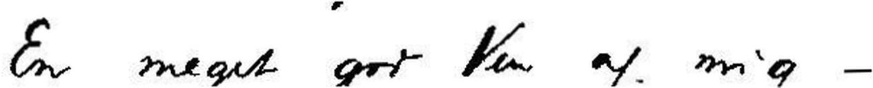En meget god Ven af mig -
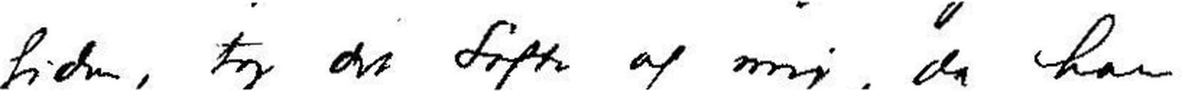siden, tog det Løfte af mig, da han
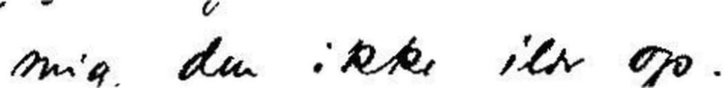mig den ikke ilde op.
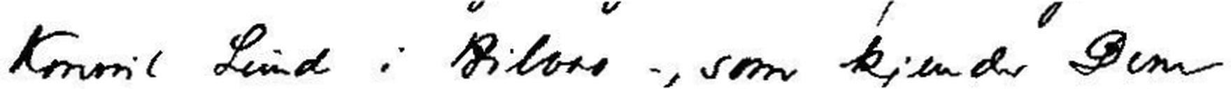Konsul Lund i Bilbao - , som kjender Dem
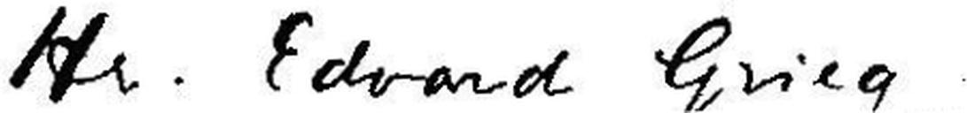Hr. Edvard Grieg.
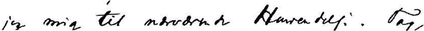jeg mig til nærværende Henvendelse. Tag
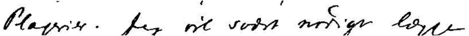Plagerier. Jeg vil svært nødigt lægge
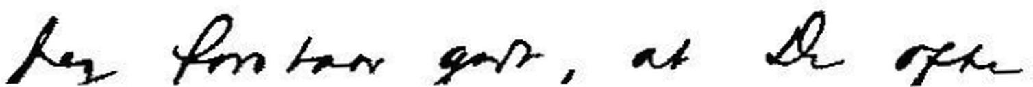Jeg forstaar godt, at De ofte
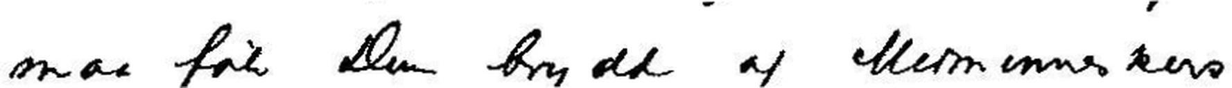maa føle Dem brydd af Medmenneskers
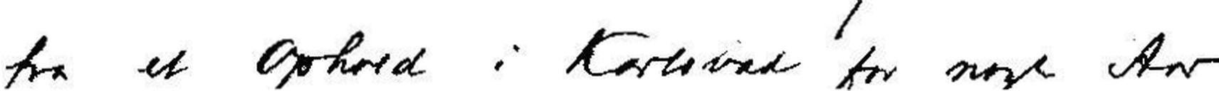fra et Ophold i Karlsbad for nogle Aar
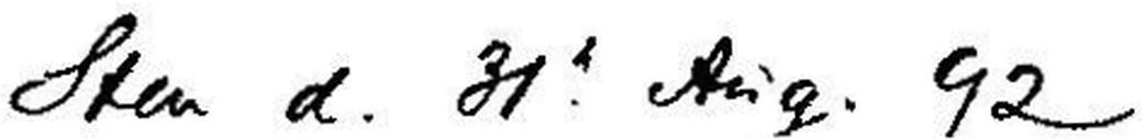Sten d. 31te Aug. 92
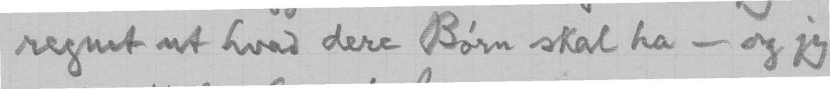regnet ut hvad dere Børn skal ha - og jeg
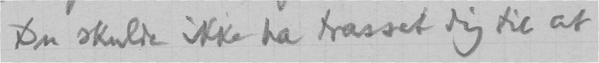Du skulde ikke ha trasset dig til at
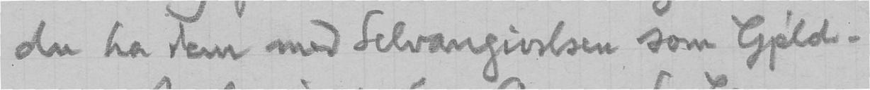du ha dem med Selvangivelsen som Gjeld.
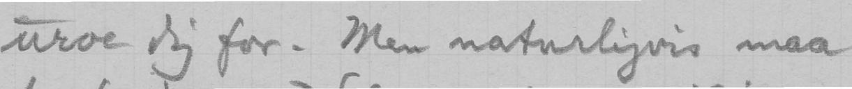uroe dig for. Men naturligvis maa
